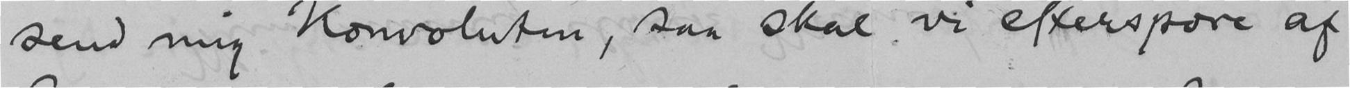send mig Konvoluten, saa skal vi efterspore af
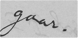gaar.
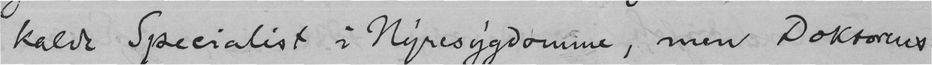kalde Specialist i Nyresygdomme, men Doktorens
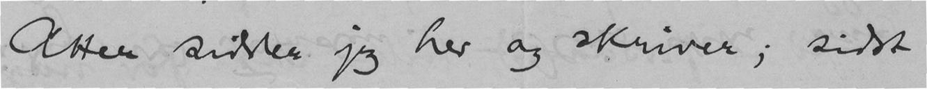Atter sidder jeg her og skriver; sidst
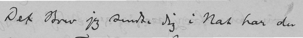Det Brev jeg sendte dig i Nat har du
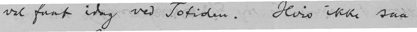vel faat idag ved Totiden. Hvis ikke saa
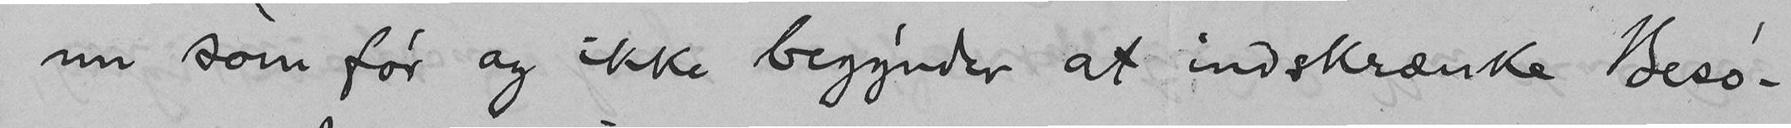nu som før og ikke begynder at indskrænke Besø-
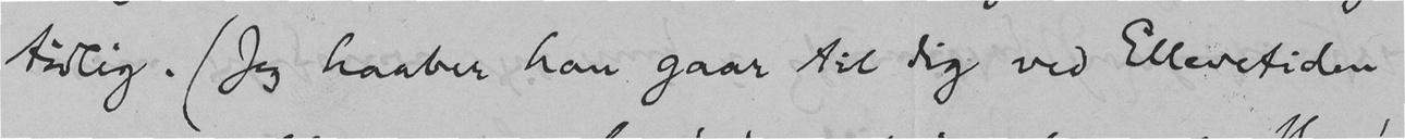tidlig. (Jeg haaber han gaar til dig ved Ellevetiden
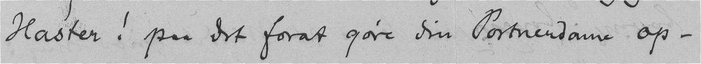Haster! paa det forat gøre din Portnerdame op-
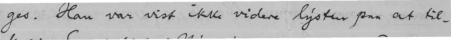ges. Han var vist ikke videre lysten paa at til-
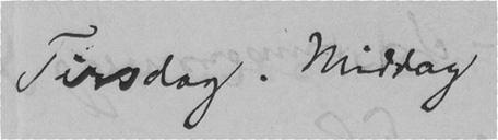Tirsdag. Middag
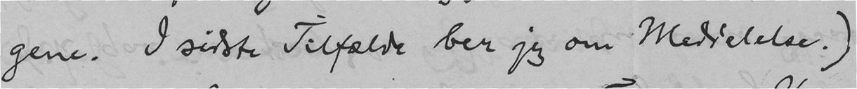gene. I sidste Tilfælde ber jeg om Meddelelse.)
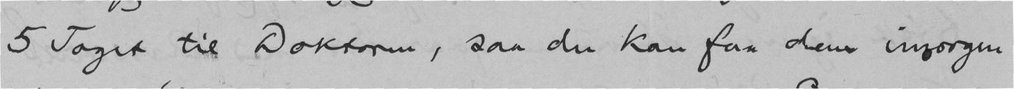5 Toget til Doktoren, saa du kan faa dem imorgen
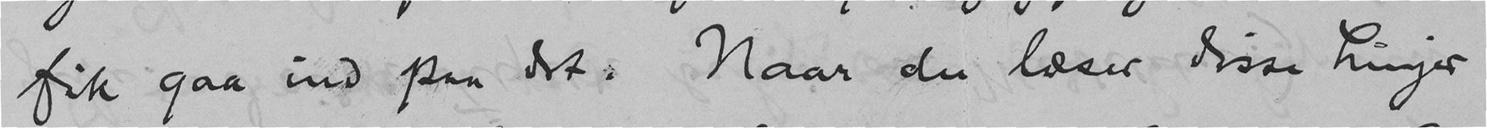fik gaa ind paa det. Naar du læser disse Linjer
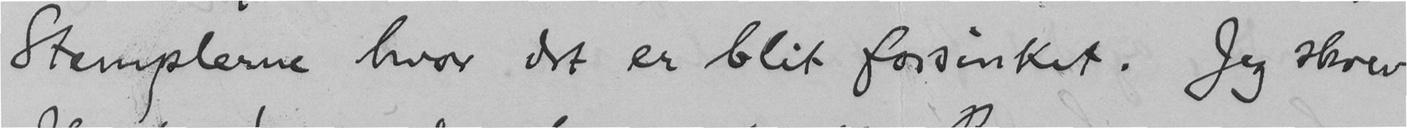Stemplerne hvor det er blit forsinket. Jeg skrev
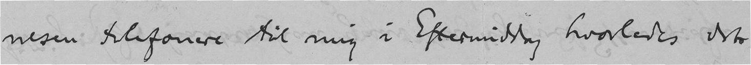nesen telefonere til mig i Eftermiddag hvorledes det
